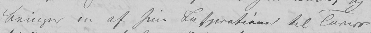bringer en af sine Inspirationer til Torvs,
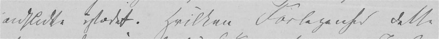udslidte istædet. Hvilken Forlegenhed dette
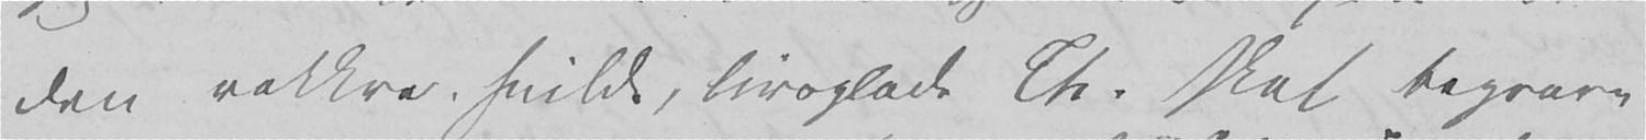den vakkre, snilde, livsglade Ch. skal begrave
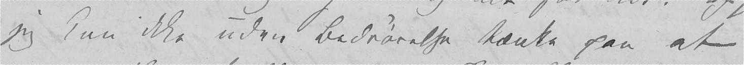jeg kan ikke uden Bedrøvelse tænke paa at
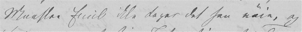Maaskee Emil ikke tager det saa nøie, og
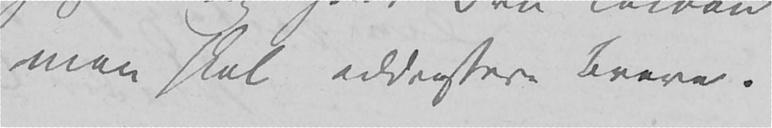man skal addressere Breve.
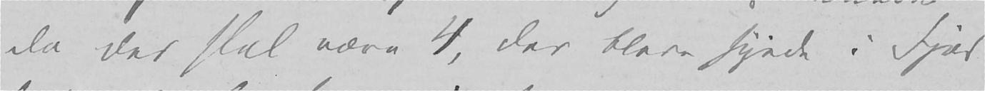da der skal være 4, der bleve syede i Fjor
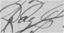Dag
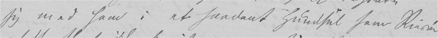sig med ham i et saadant Hundhul som Riesør
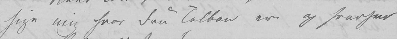sige mig hvor Fru Colban er og hvorhen
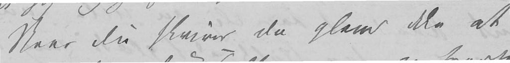Naar Du skriver da glem ikke at
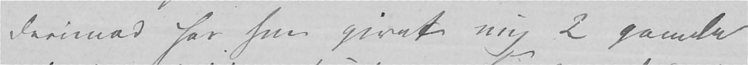derimod har hun givet mig 2 gamle
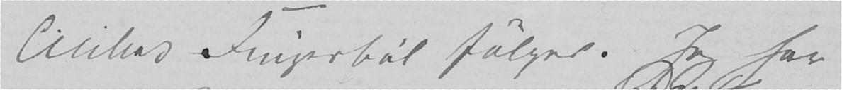Cicilies Fingerbøl følger. Jeg har
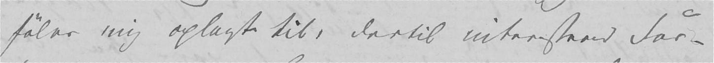føler mig oplagt til, dertil interesserer For-
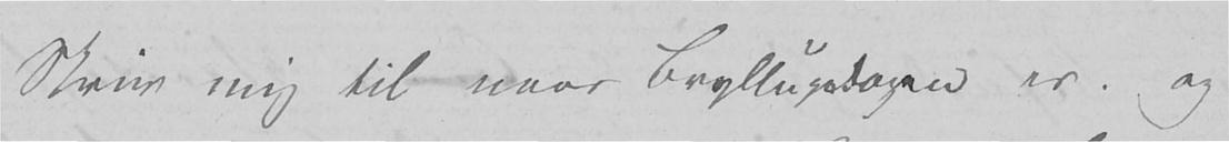Skriv mig til naar Bryllupsdagen er. og
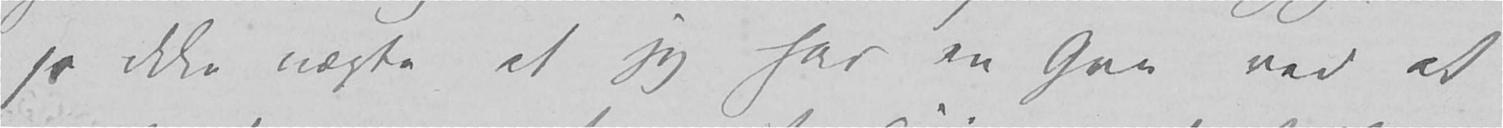jo ikke nægte at jeg har en Gru ved at
